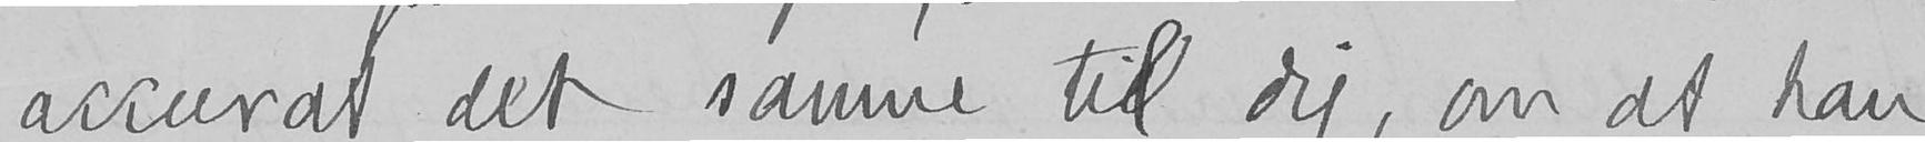accurat det samme til dig, om at han
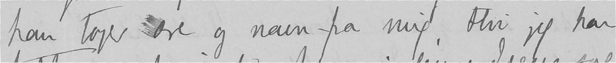han tager ære og navn fra mig, thi jeg har
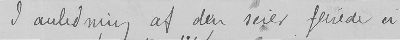I anledning af den seier feirede vi
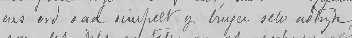ens ord saa simpelt og bruger selv udtryk,
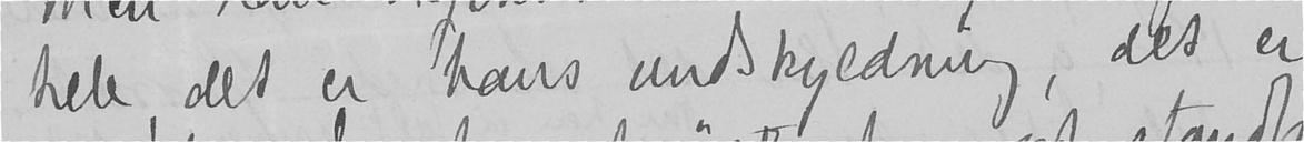hele, det er hans undskyldning, det er
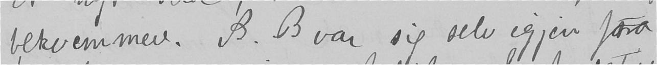bekvemmere. B. B var sig selv igjen fra
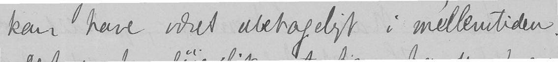kan have været ubehageligt i mellemtiden.
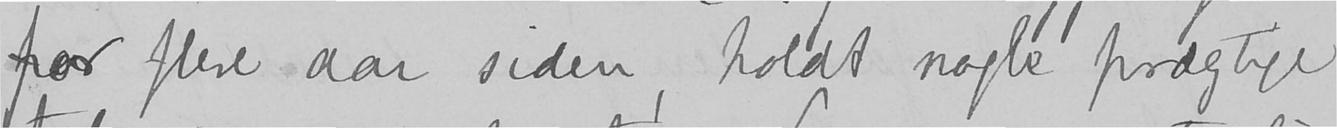for flere aar siden, holdt nogle prægtige
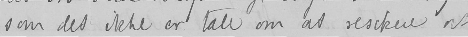som det ikke er tale om at resikere at
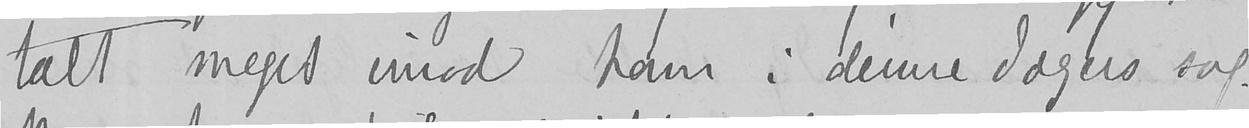talt meget imod ham i denne Jægers sag.
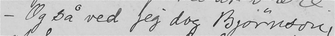- Og så ved jeg dog Bjørnson,
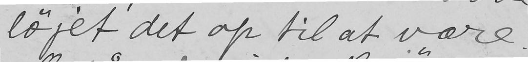løjet det op til at være.
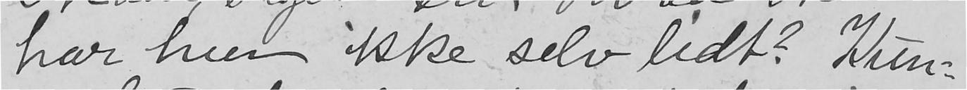har hun ikke selv lidt? Kun-
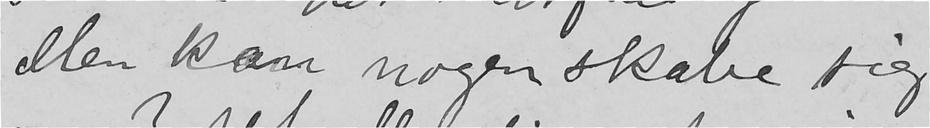Men kan nogen skabe sig
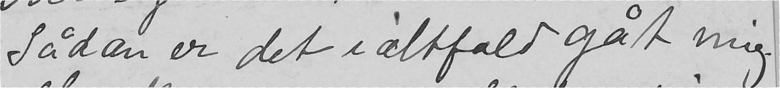Sådan er det ialtfald gåt mig
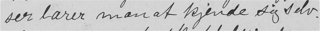ser lærer man af kjende sig selv.
